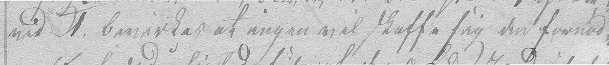ved 1. bevirkes at ingen vil skaffe sig den fornød-
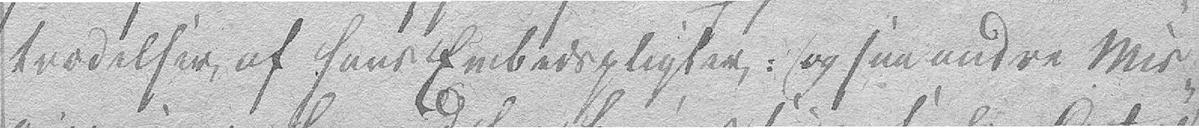trædelser af hans Embedspligter: ogsaa andre Mis-
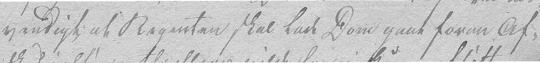vendigt at Regenten skal lade Dom gaae foran Af-
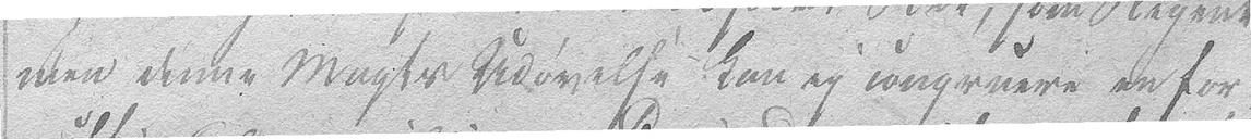men denne Magts Udøvelse kan ey congruere en for-
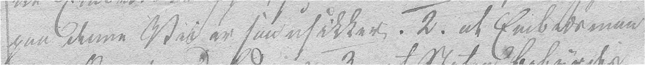paa denne Vei er saa usikker. 2. at Embedsman-
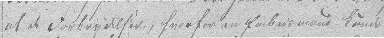at de Forbrydelser, hvorfor en Embedsmand kunde
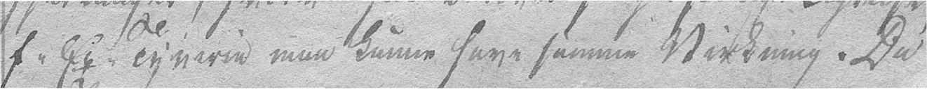f: Ex: Tyverie, maa kunne have samme Virkning. Da
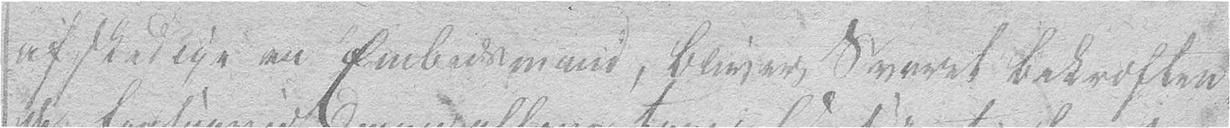afskedige en Embedsmand, bliver Svaret bekræften-
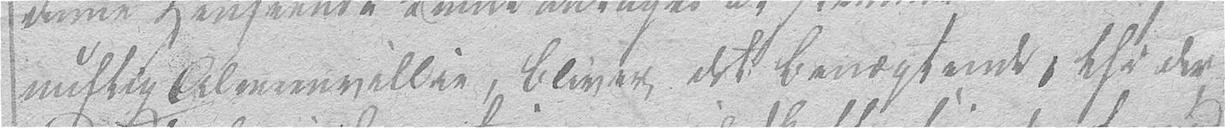nuftig Almeenvillie, bliver det benægtende, thi der-
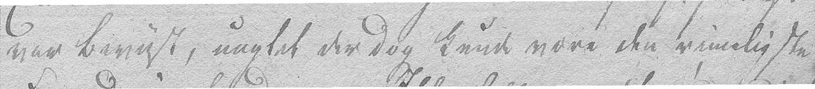var beviist, uagtet der dog kunde være den rimeligste
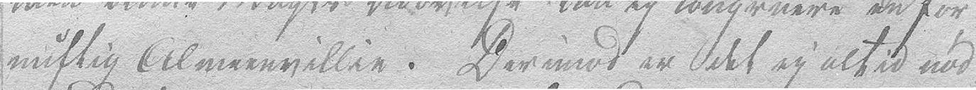nuftig Almeenvillie. Derimod er det ey altid nød-
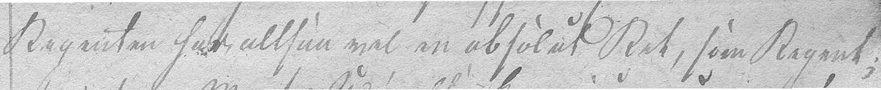Regenten har altsaa vel en absolut Ret, som Regent;
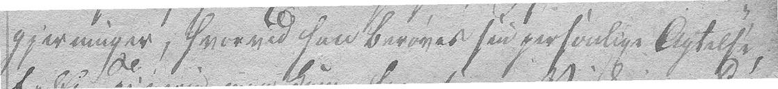gjerninger, hvorved han berøves sin personlige Agtelse,
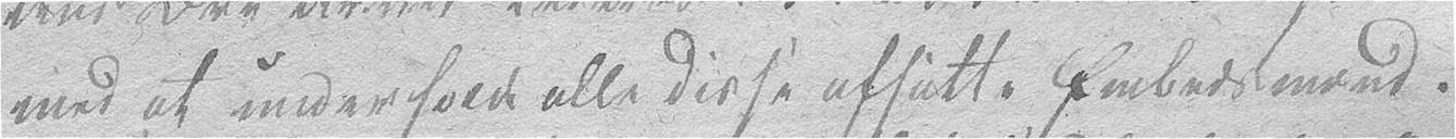med at underholde alle disse afsatte Embedsmænd.
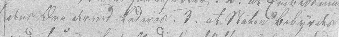dens Ære derved laderes. 3. at Staten bebyrdes
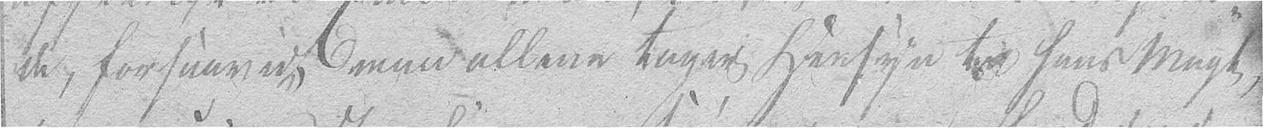de, forsaavidt man allene tager Hensyn til hans Magt,
